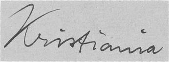Kristiania
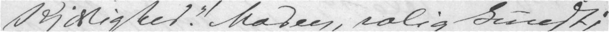Kjærlighed"! Moden, rolig kunst;
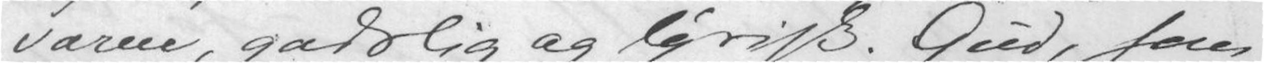varm, godslig og lyrisk. Gud, som
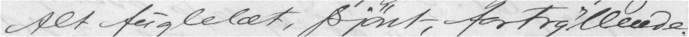Alt fuglelæt, skjønt, fortryllende.
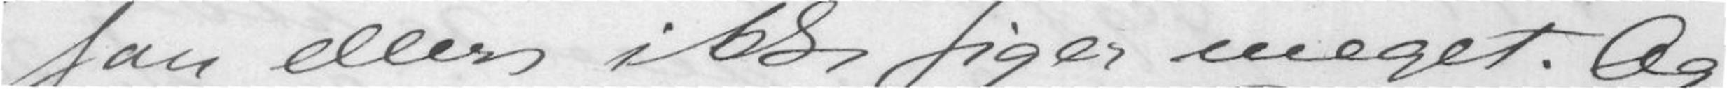som ellers ikke siger meget. Og
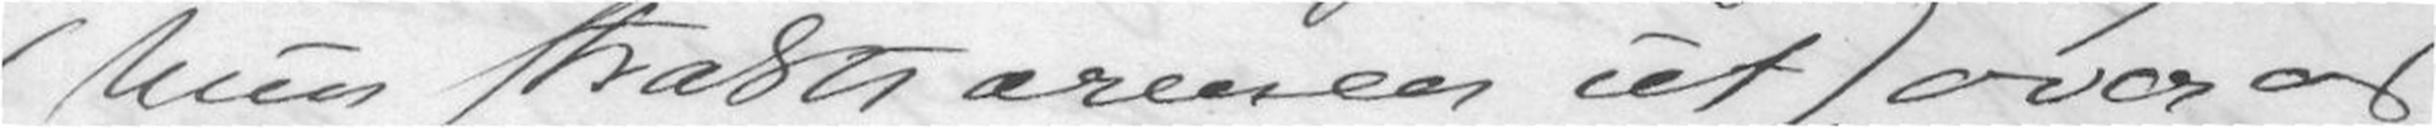(hun strakte armen ut) over os
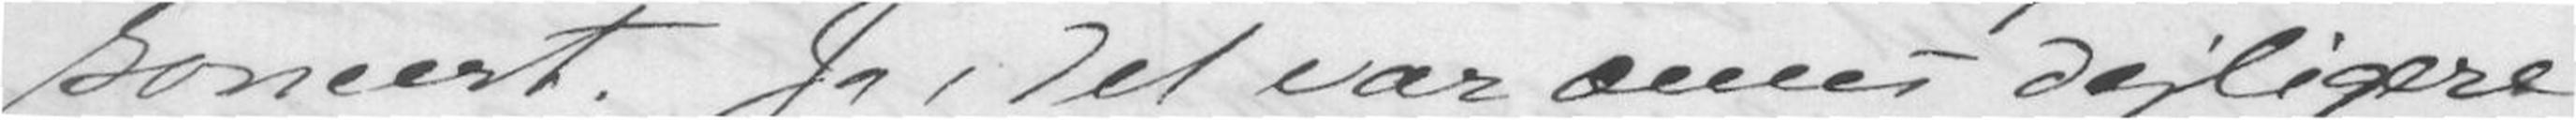koncert. Ja, det var ænnu dejligere
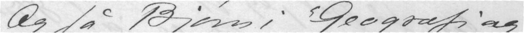Og så Bjørn i "Geografi og
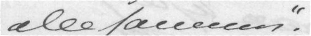allesammen.
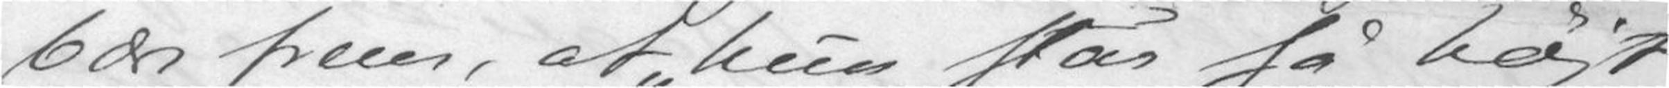bære frem, at hun står så højt
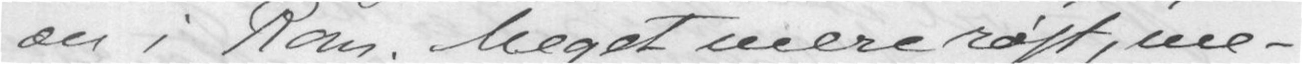æn i Rom. Meget mere røst, me-
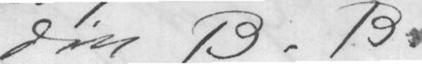Din B. B.
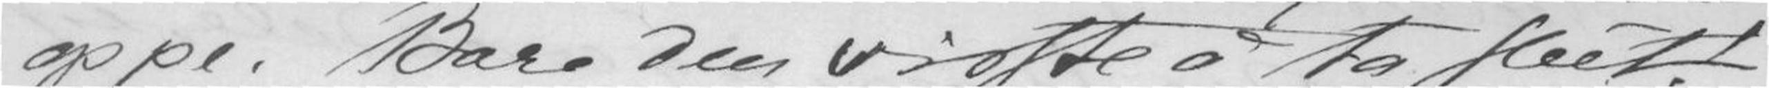oppe. Bare den visste å ta slut!
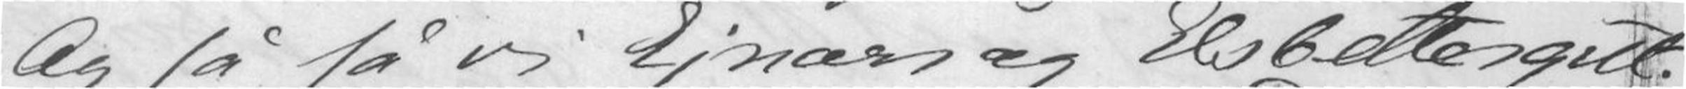Og så vi Ejnars og Elsbettes gut.
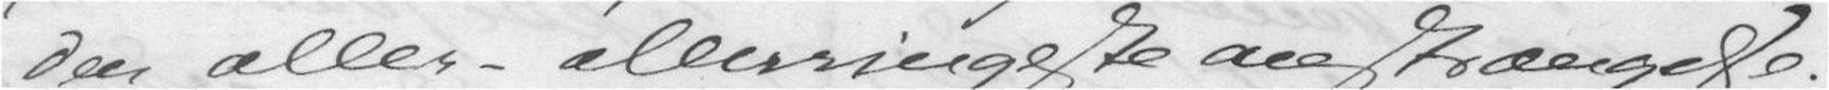den aller-allerringeste anstrængelse.
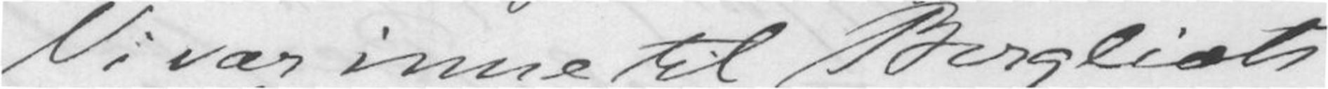Vi var inne til Bergliots
